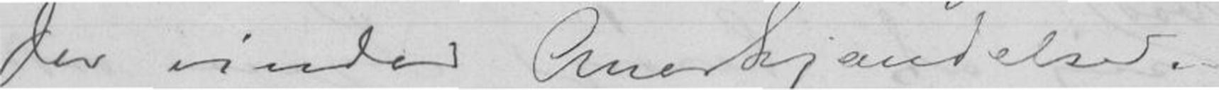du vinder Anerkjændelse.
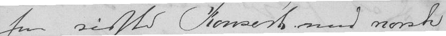sin sidste Konsert med norsk
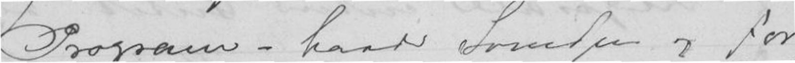Program. baade Svendsen og For
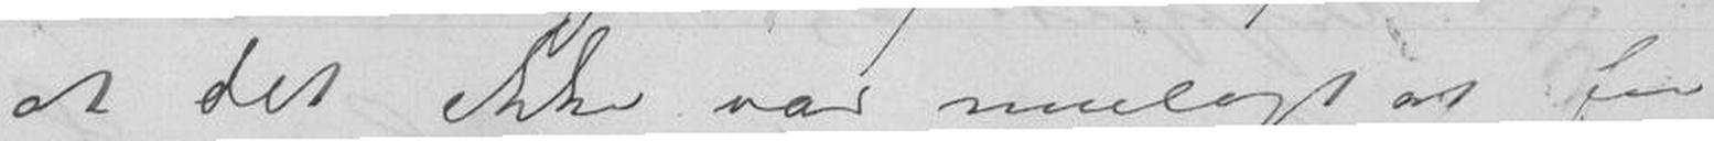at det ikke var muligt at faa
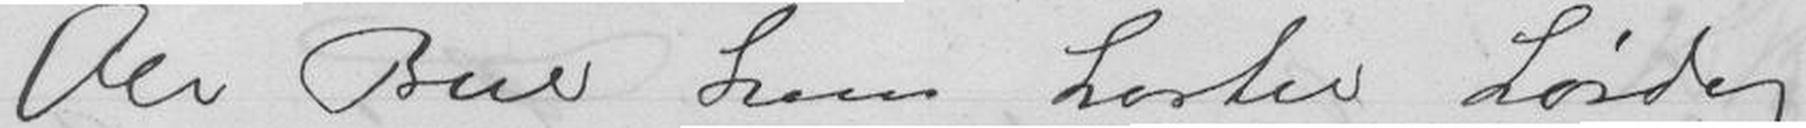Ole Bul kom hertil Lørdag
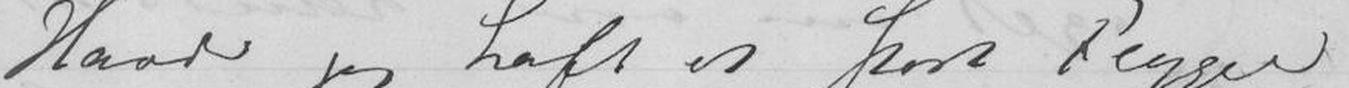Havde jeg haft et stort Flygel
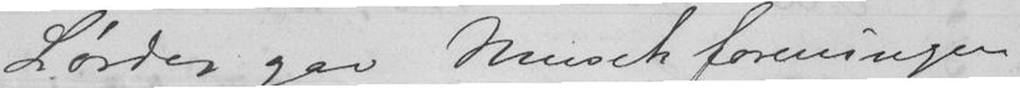Lørdag gav Musikforeningen
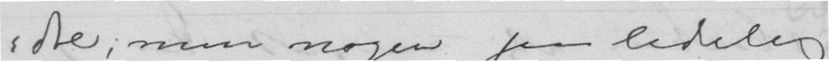dre, men nogen paalidelig
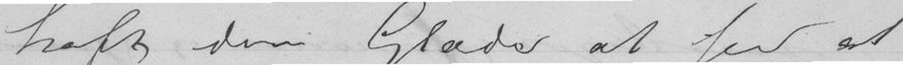haft den Glæde at see at
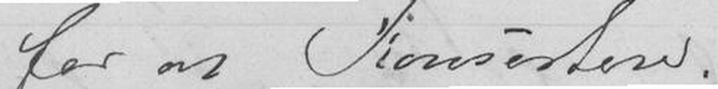for at Konsertere.
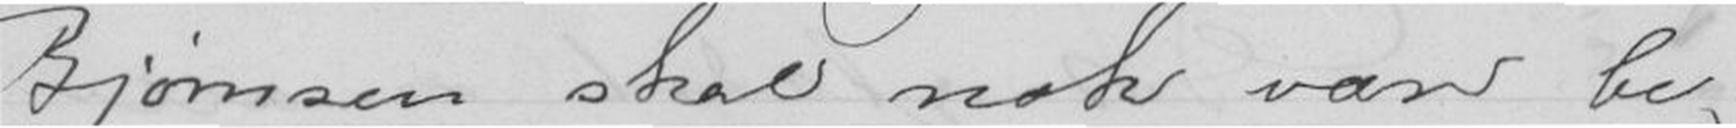Bjørnson skal nok være be
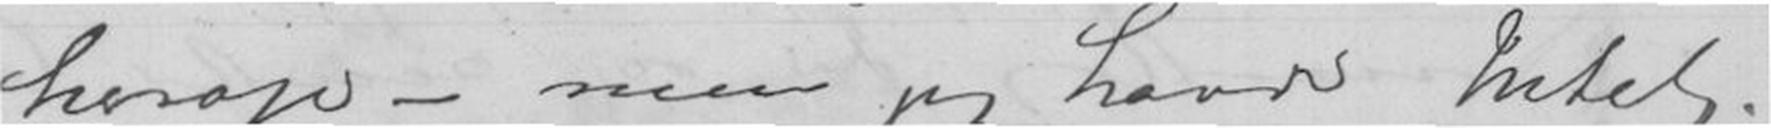herop - men jeg havde Intet.
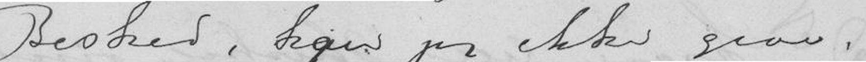Besked, kan jeg ikke give.
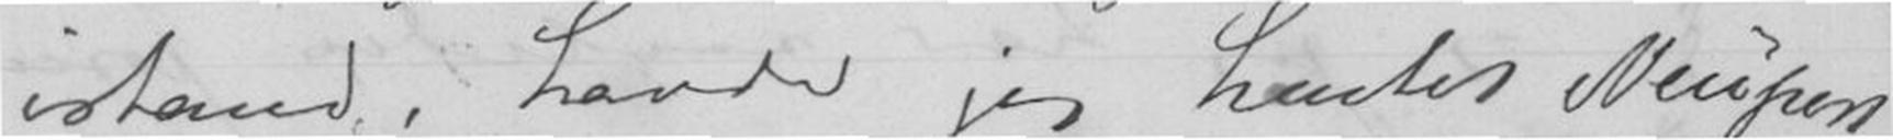istand, havde jeg hæntet Neupert
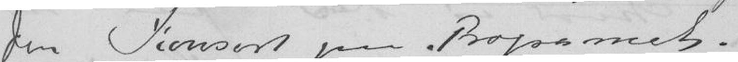din Konsert paa Programet.
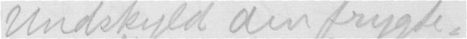Undskyld den frygte-
